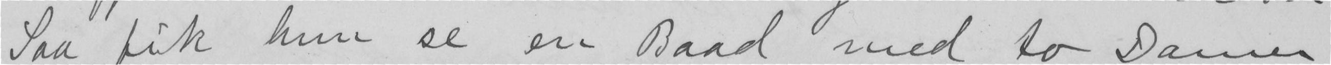Saa fik hun se en Baad med to Damer
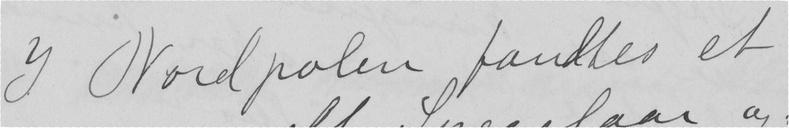I Nordpolen fandtes et
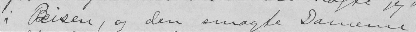i Peisen, og den smagte Damerne
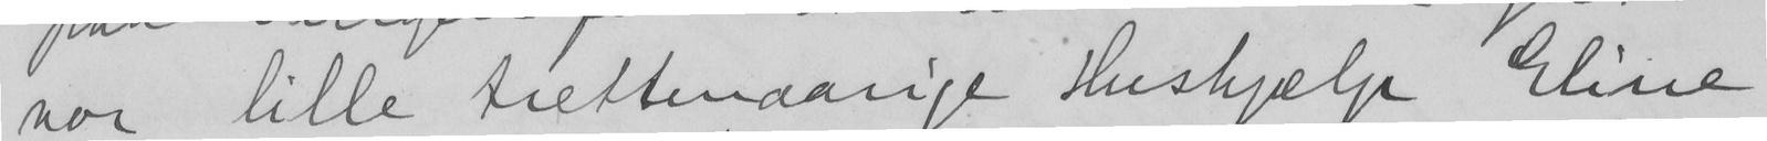vor lille trettenaarige Hushjælp Eline
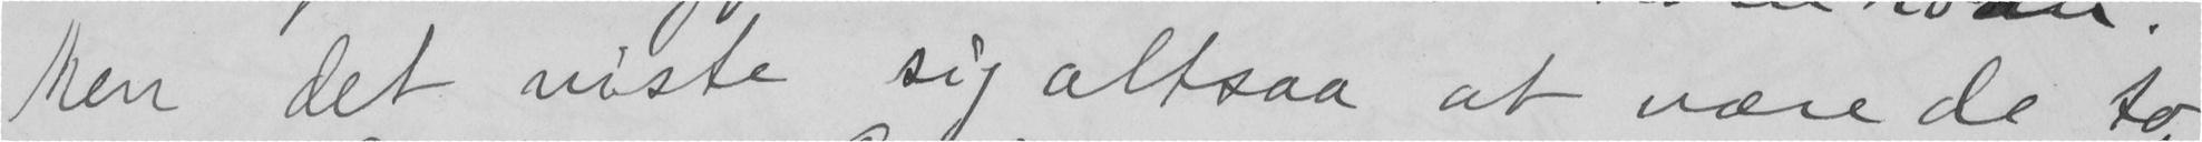Men det viste sig altsaa at være de to
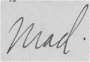Mad.
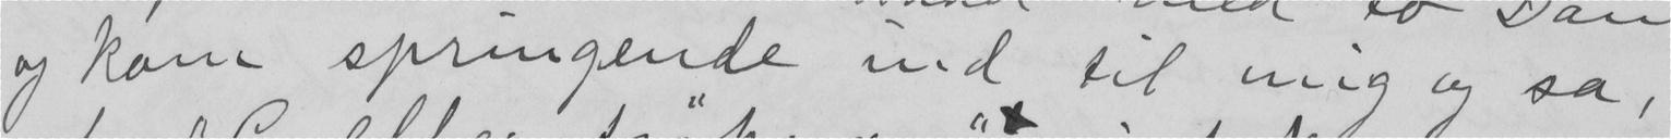og kom springende ind til mig og sa,
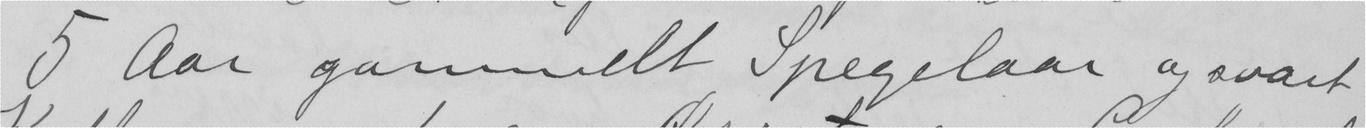5 Aar gammelt Spegelaar og svart
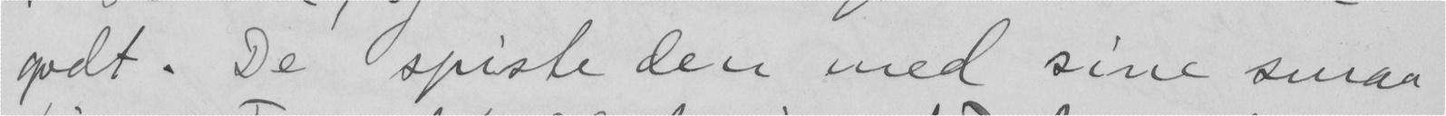godt. De spiste den med sine smaa
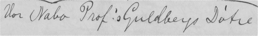Vor Nabo Prof.'s Guldbergs Døtre
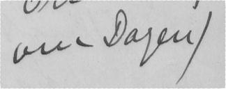om Dagen)
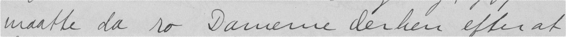maatte da ro Damerne derhen efter at
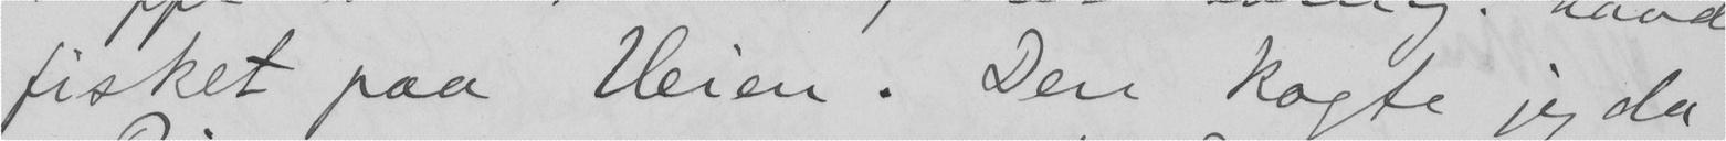fisket paa Veien. Den kogte jeg da
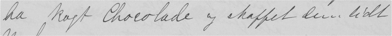ha kogt Chocolade og skaffet dem lidt
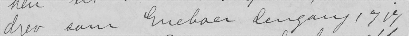drev som Eneboer dengang, og jeg
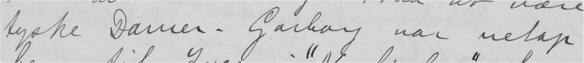tyske Damer. Garborg var netop
